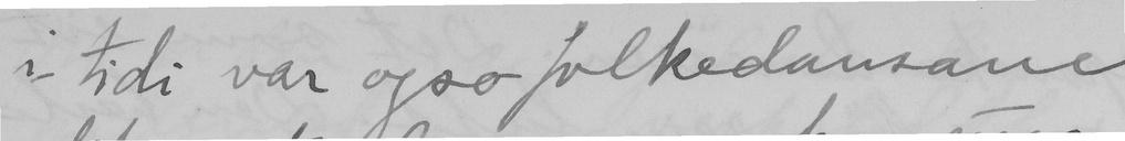i tidi var ogso folkedansane
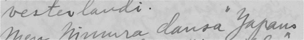Men nimmra dansa "Japans
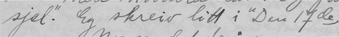sjel". Eg skreiv litt i "Den 17de".
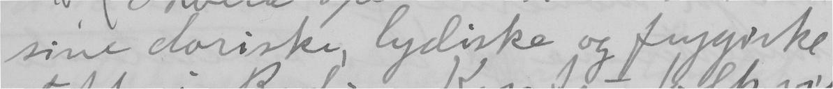sine doriske, lydiske og fuggiske
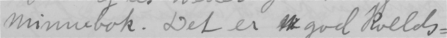minnebok. Det er god kvelds-
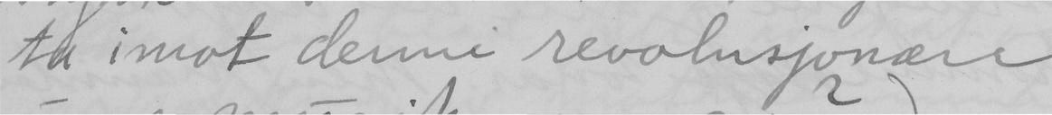ta imot denne revolusjonære
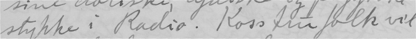stykke i Radio. Koss tru folk vil
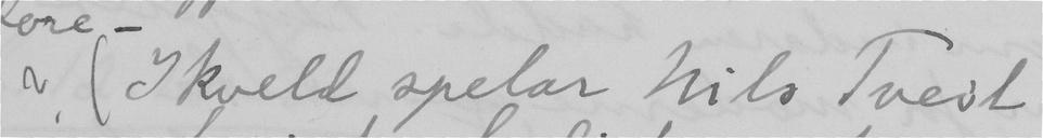Ikveld spelar Nils Tveit
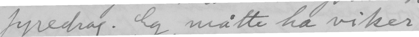fyredrag. Eg måtte ha viker
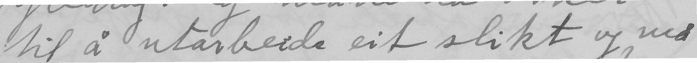til å utarbeide eit slikt og må
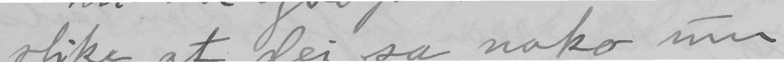slike at dei sa noko um
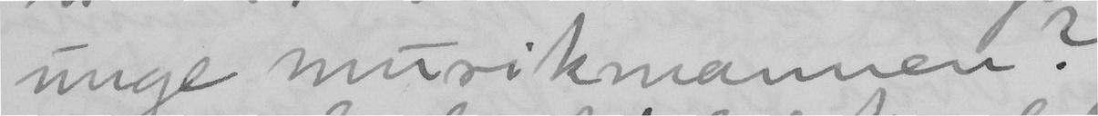unge musikmannen?
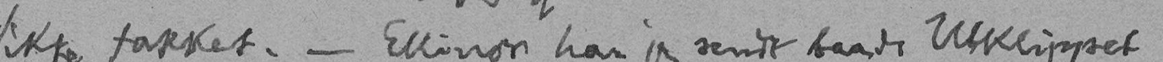ikke takket. - Ellinor har jeg sendt baade Utklippet
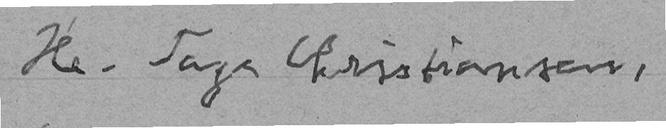Hr. Tage Christiansen,
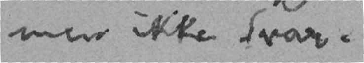men ikke Svar.
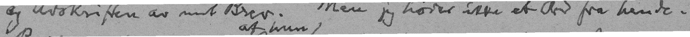og Avskriften av mit Brev. Men jeg hører ikke et Ord fra hende.
